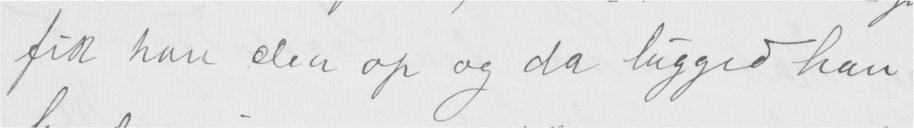fik han den op og da lugged han
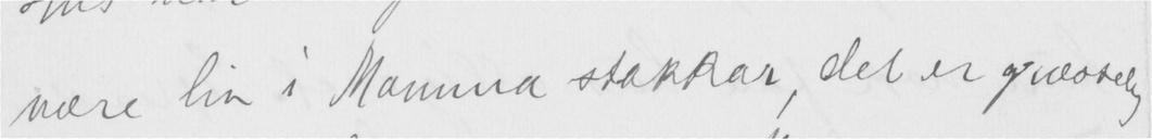være liv i Mamma stakkar, det er græsselig
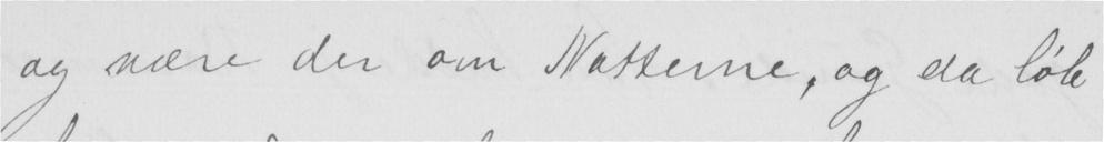og være der om Nætterne, og da løb
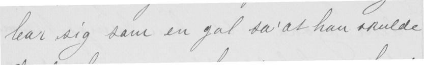bar sig som en gal sa'at han skulde
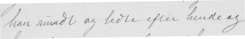han rundt og ledte efter hende og
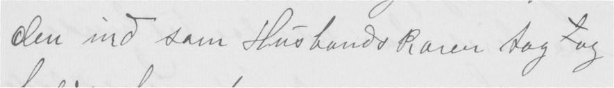den ind som Husbondskaren tog tag
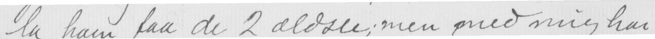la ham faa de 2 ældste; men med mig har
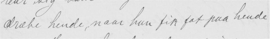dræbe hende, naar han fik fat paa hende
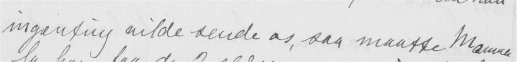ingenting vilde sende os, saa maatte Mamma
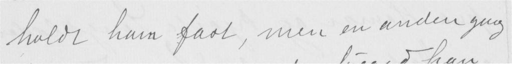holdt ham fast, men en anden gang
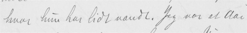hvor hun har lidt vondt. Jeg var et Aar
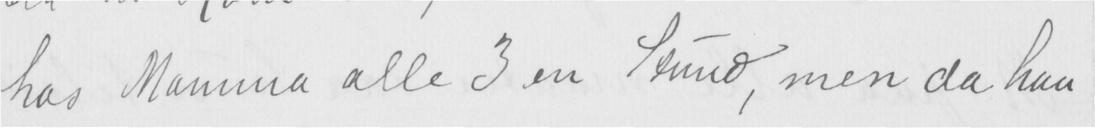hos Mamma alle 3 en stund, men da han
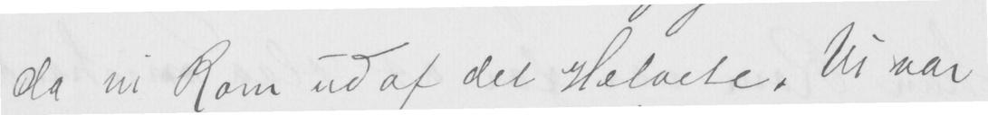da vi kom ud af det Helvete. Vi var
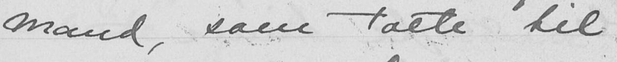mand, som talte til
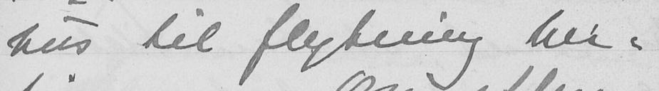hus til flytning her-
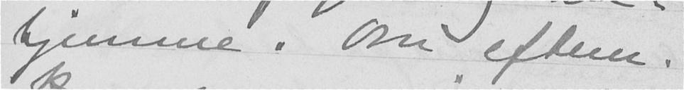hjemme. Om efterm.
